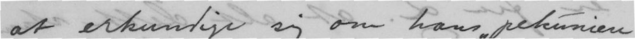at erkundige sig om hans "pekuniere
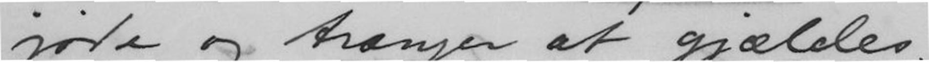jöde og trænger at gjældes.
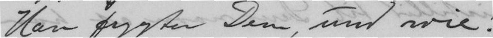Han frygter Dem, und wie!
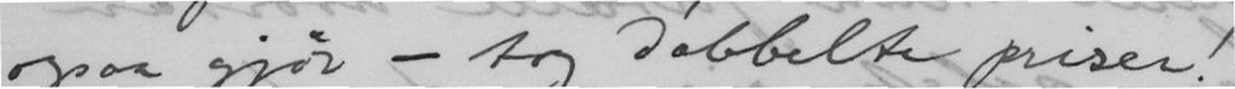ogsaa gjör - tog dobbelte priser!
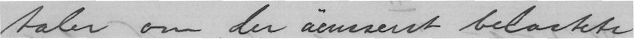taler om "der aeusserst belastete
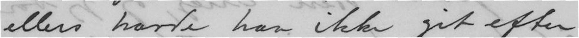ellers havde han ikke git efter
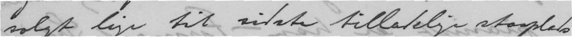solgt lige til sidste tilladelige staaplads,
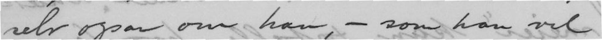selv ogsaa om han - som han vel
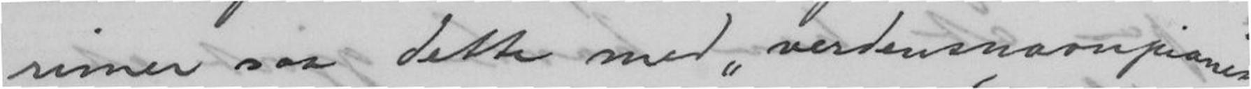rimer saa dette med "verdensnavnpianisten"?!
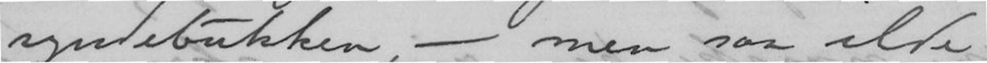syndebukken,- men saa ilde
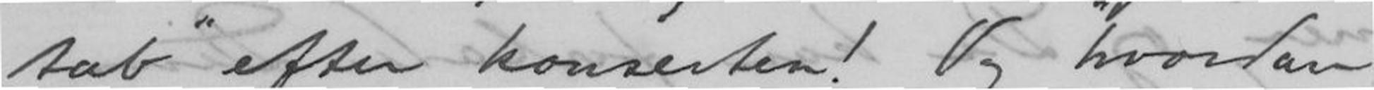tab" efter konserten! Og hvordan
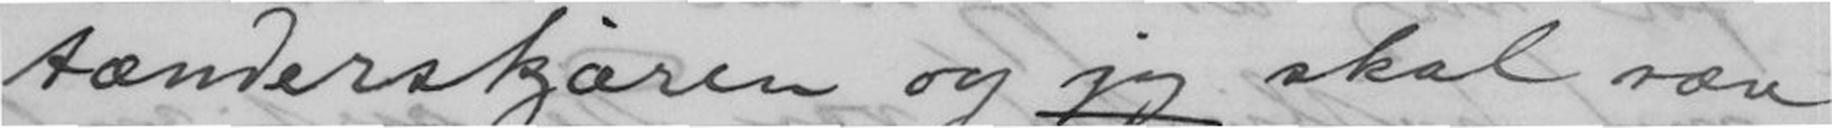tænderskjæren og jeg skal være
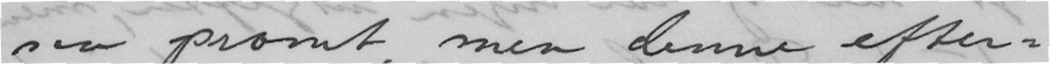saa prompt, men denne "efter-
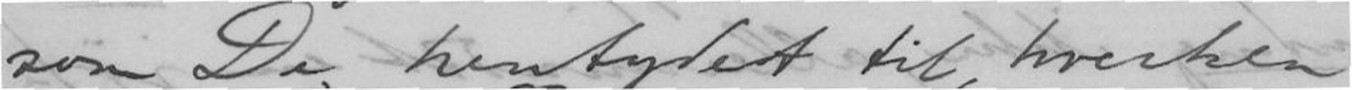som De hentydete til, hverken
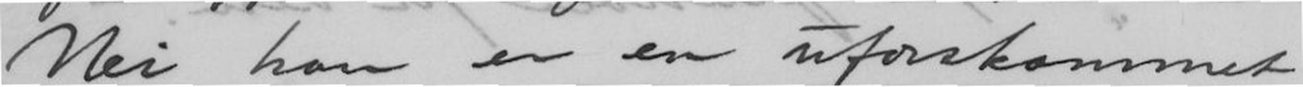Nei han er en uforskammet
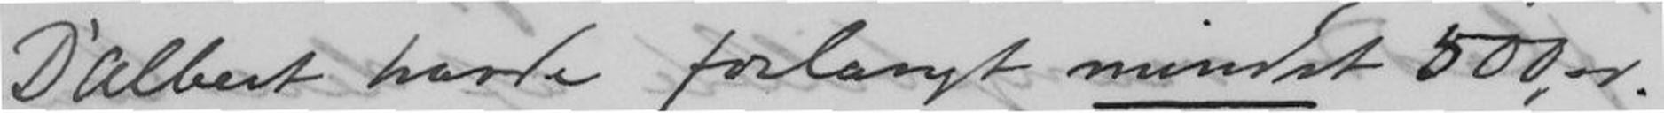D'Albert havde forlangt mindst 500,00.
